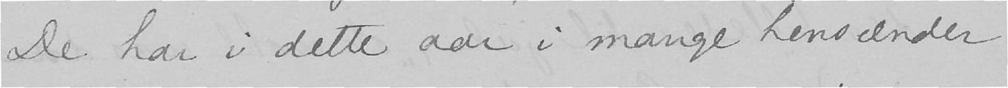De har i dette aar i mange henseender
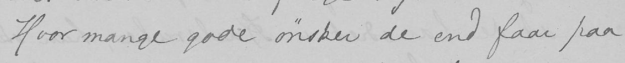Hvor mange gode ønsker de end faar paa
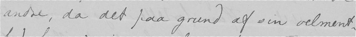andre, da det paa grund af sin velment-
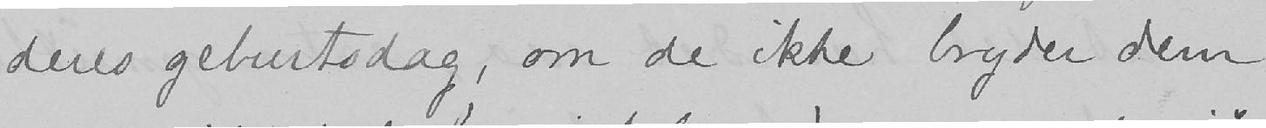deres geburtsdag, om de ikke bryder dem
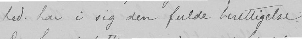hed har i sig den fulde berettigelse.
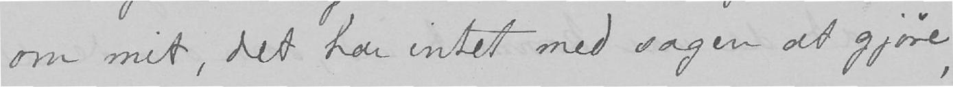om mit, det har intet med sagen at gjøre,
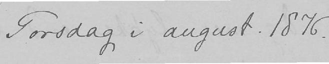Torsdag i august. 1876.
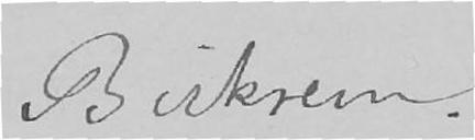Birkrem.
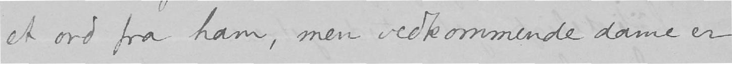et ord fra ham, men vedkommende dame er
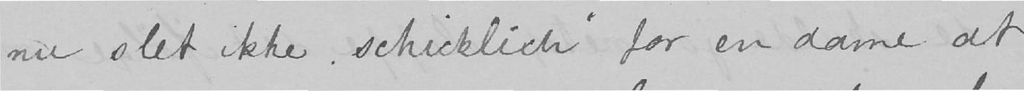nu slet ikke «schicklich» for en dame at
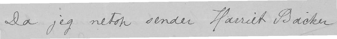Da jeg netop sender Harriet Backer
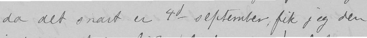da det snart er 4de september, fik jeg den
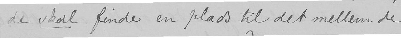de skal finde en plads til det mellem de
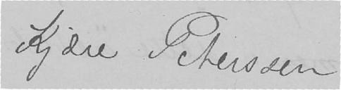Kjære Peterssen
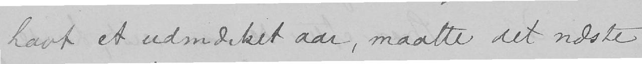havt et udmærket aar, maatte det næste
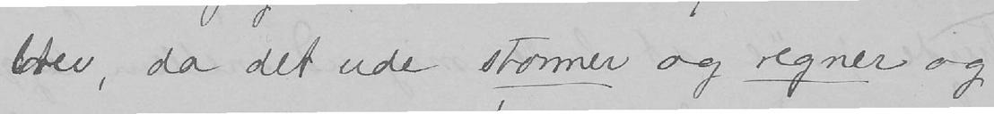brev, da det ude stormer og regner og
